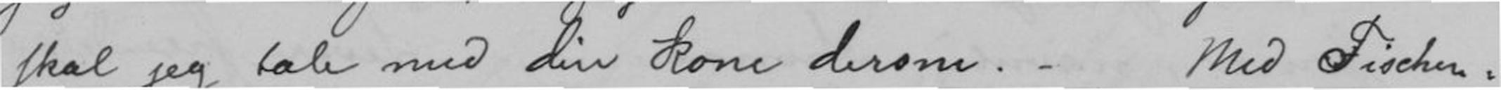skal jeg tale med din kone dersom. - Med Tischen-
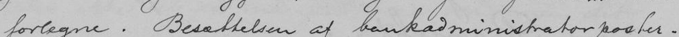forlegne. Besættelsen af bankadministratorposter-
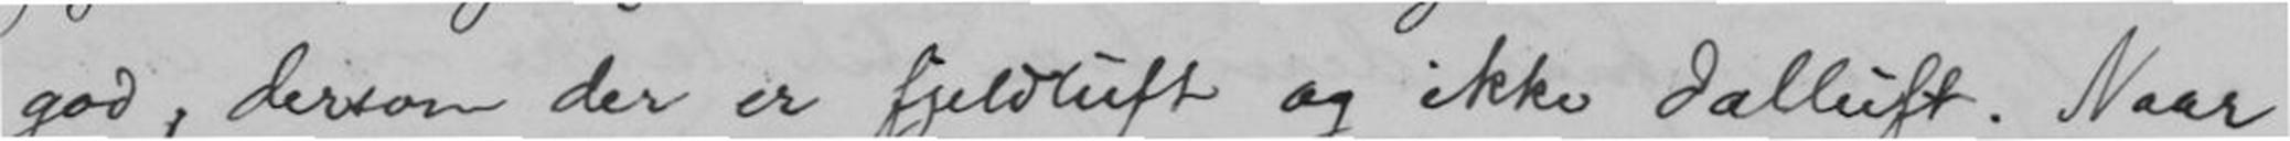god, dersom der er fjeldluft og ikke dalluft. Naar
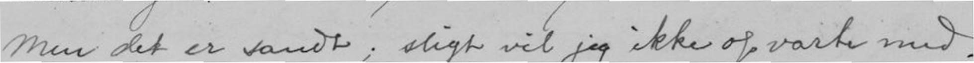Men det er sandt; sligr vil jeg ikke opvarte med.
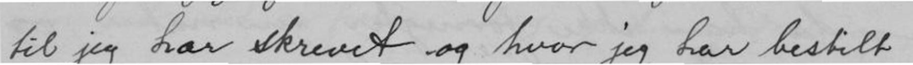til jeg har skrevet og hvor jeg har bestilt
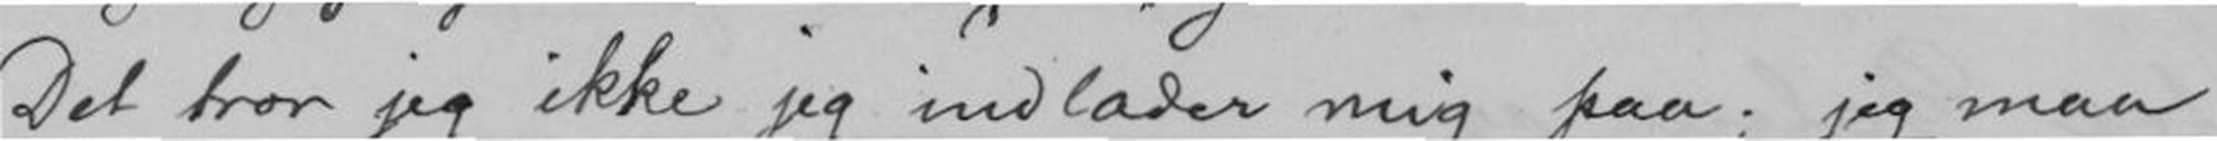Det tror jeg ikke jeg indlader mig paa; jeg maa
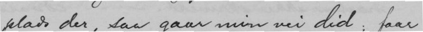plads der, saa gaar min vei did; faar
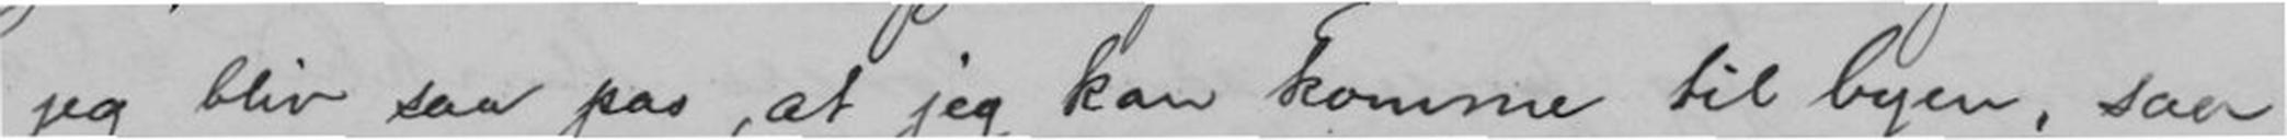jeg blir saa pas, at jeg kan komme til byen, saa
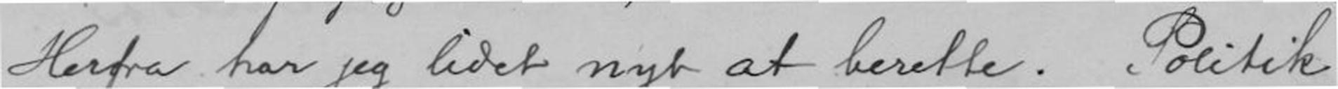Herfra har jeg lidet nyt at berette. Politik
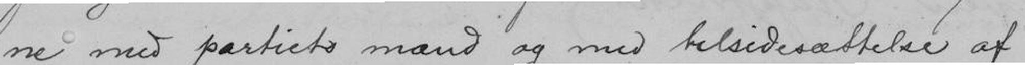ne med partiets mænd og med tilsidesættelse af
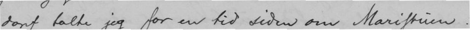dorf talte jeg for en tid siden om Maristuen.
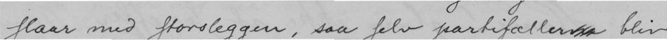slaar med storsleggen, saa selv partifæller blir
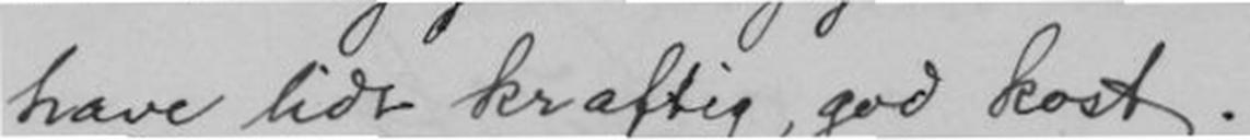have lidt kraftig god kost.
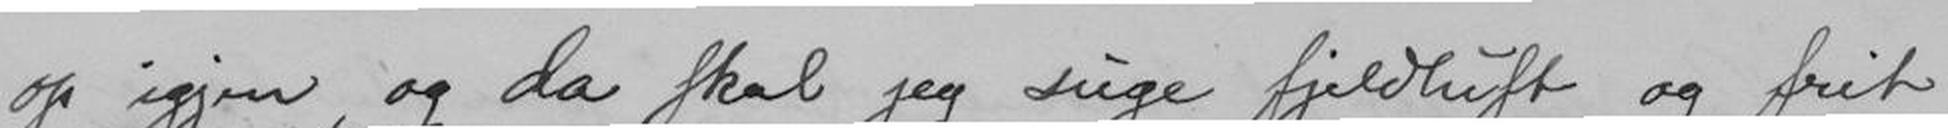op igjen, og da skal jeg suge fjeldluft og frit
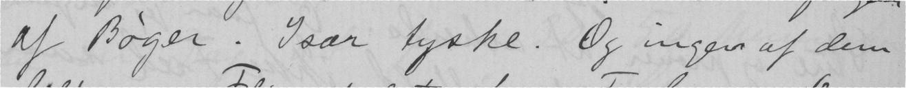af Bøger. Især tyske. Og ingen af dem
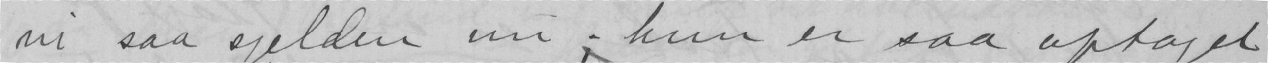vi saa sjelden nu; hun er saa optaget
